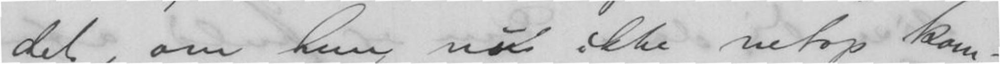det, om hun nu ikke netop kom-
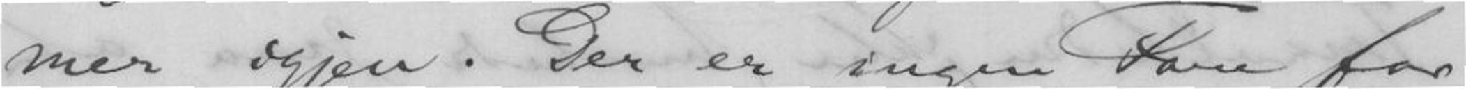mer igjen. Der er ingen Fare for
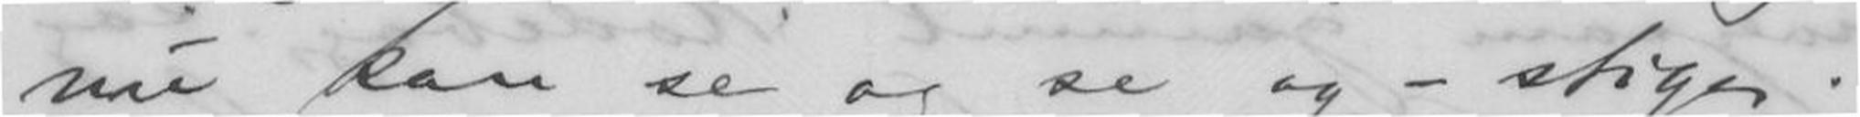nu kan se og se og - stige.
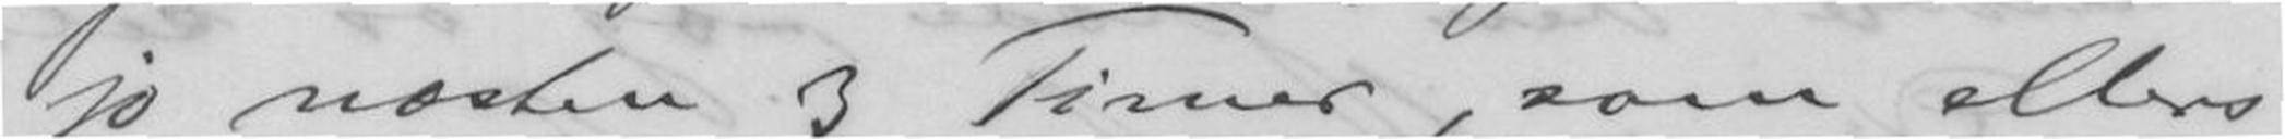jo næsten 3 Timer, som ellers
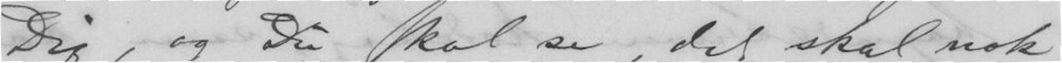Dig, og Du skal se, det skal nok
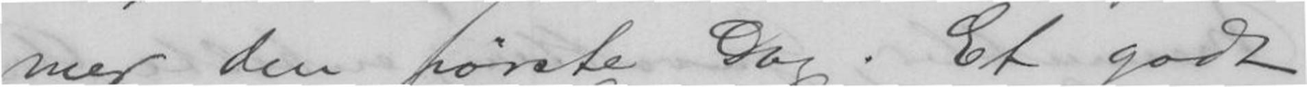mer den første Dag. Et godt
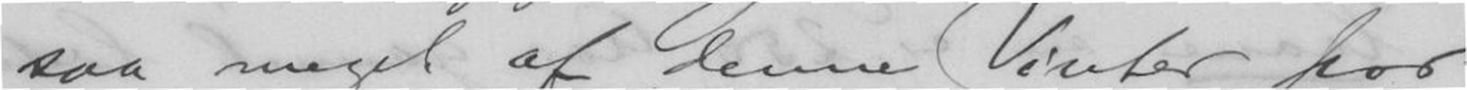saa meget af denne Vinter for
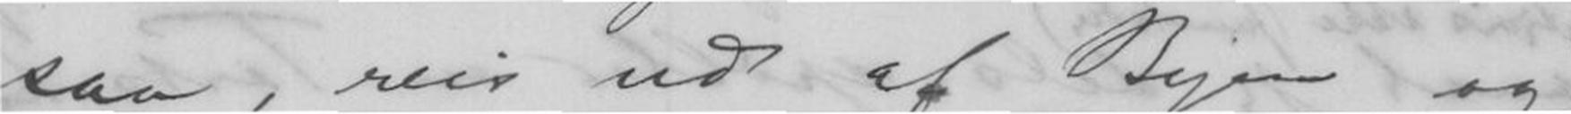saa, reis ud af Byen og
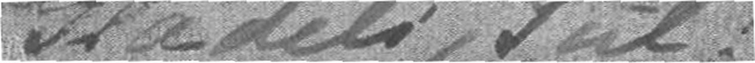Glædelig Jul!
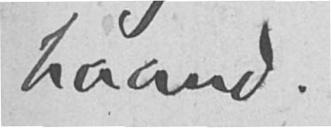haand.
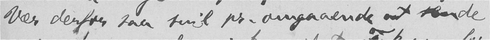Vær derfor saa snil pr. omgaaende at sende
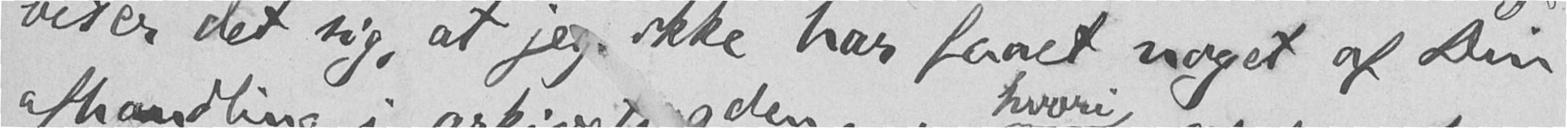viser det sig, at jeg ikke har faaet noget af Din
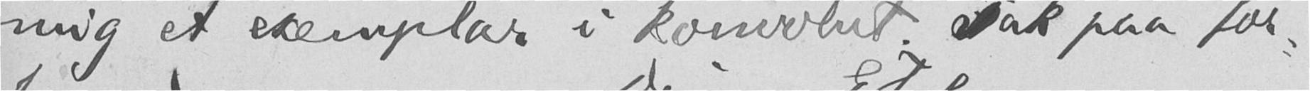mig et exemplar i konvolut. Tak paa for-
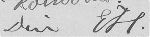Din EH.
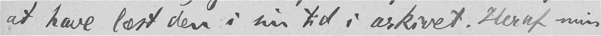at have læst den i sin tid i arkivet. Heraf min
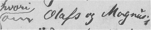om Olafs og Magnus's
